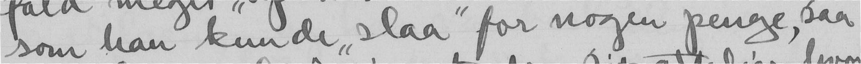som han kunde "slaa" for nogen penge, saa
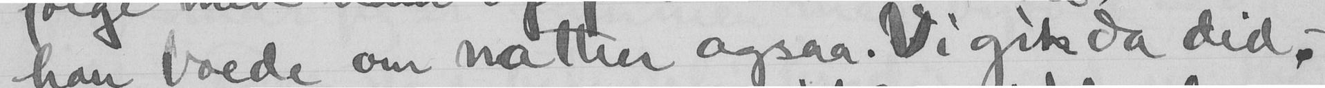han boede om natten ogsaa. Vi gik da did,-
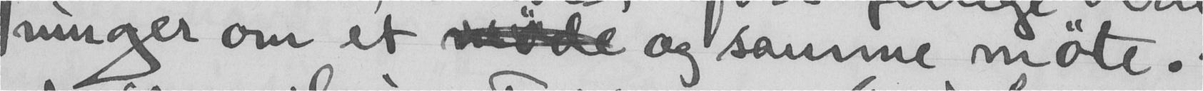ninger om et  og samme møte.
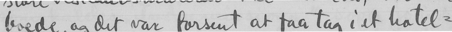boede, og det var forsent at faa tag i et hotel-
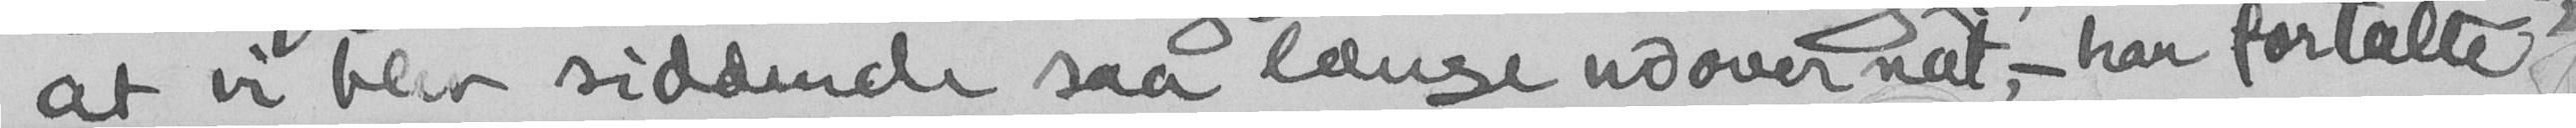at vi blev siddende saa længe udover nat, - han fortalte
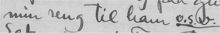min seng til ham o.s.v.
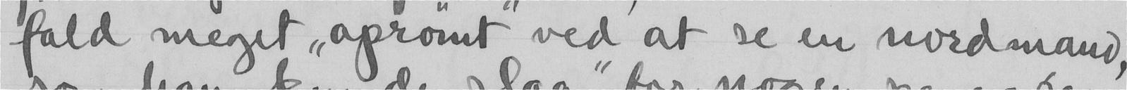fald meget "oprømt" ved at se en nordmand,
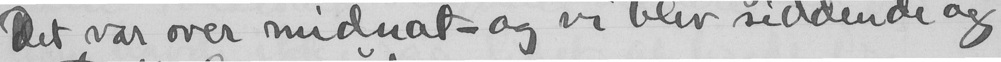det var over midnat - og vi blev siddende og
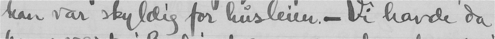han var skyldig for husleien. - Vi havde da
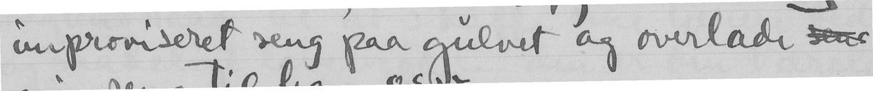improviseret seng paa gulvet og overlade
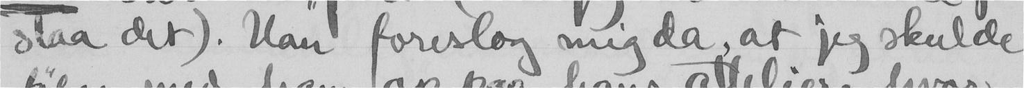staa det). Han foreslog mig da, at jeg skulde
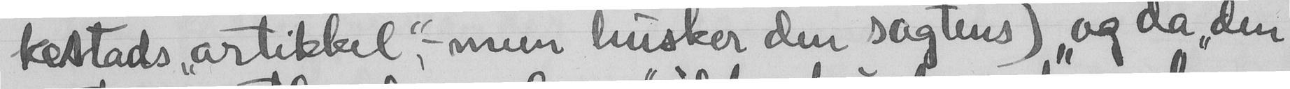kestads "artikkel", - men husker den sagtens) og da "den
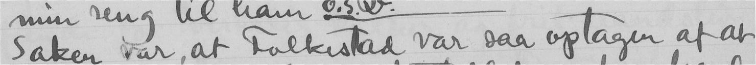Saken var, at Folkestad var saa optagen af at
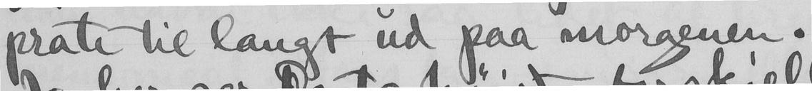prate til langt ud paa morgenen.
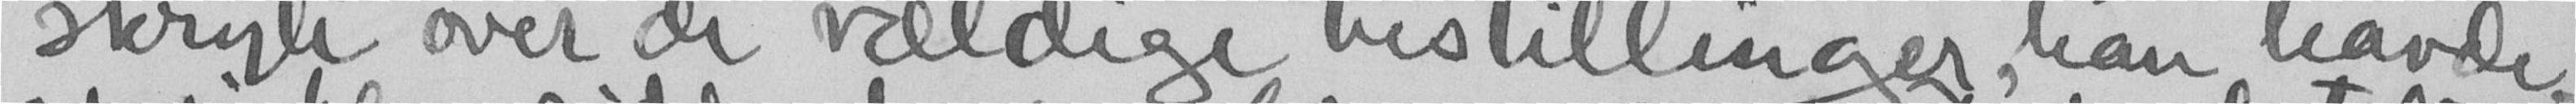skryte over de vældige bestillinger han havde
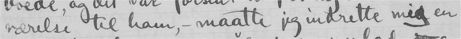værelse til ham - maatte jeg indrette med en
